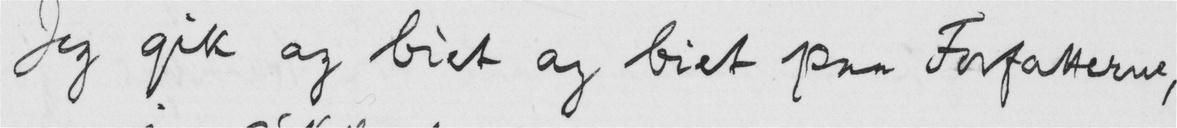Jeg gik og biet og biet paa Forfatterne,
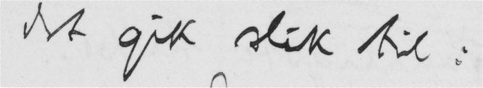det gik slik til:
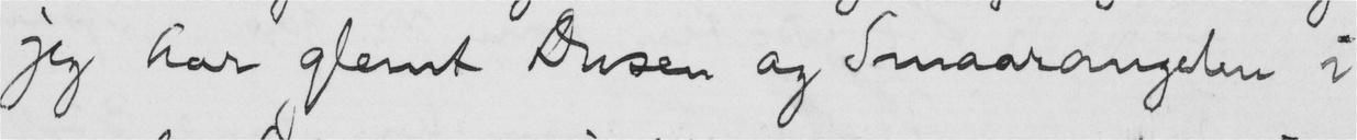jeg har glemt Dusen og Smaarangelen i
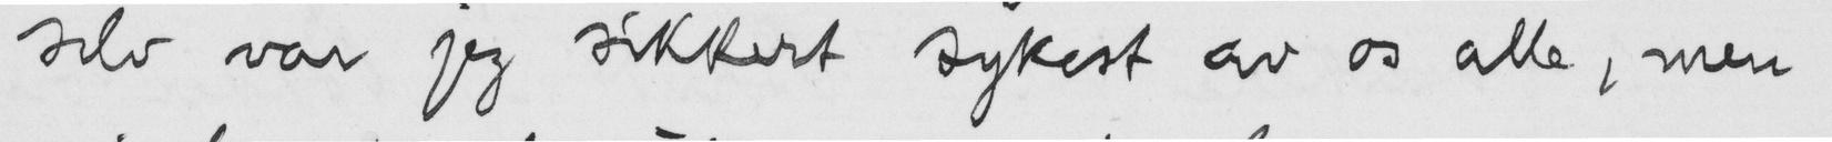selv var jeg sikkert sykest av os alle, men
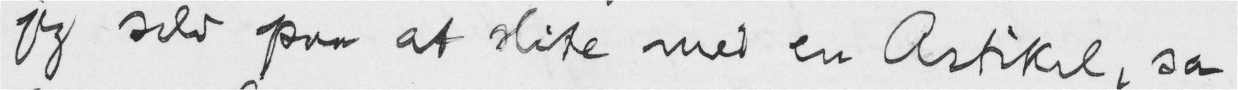jeg selv paa at slite med en Artikel, sa
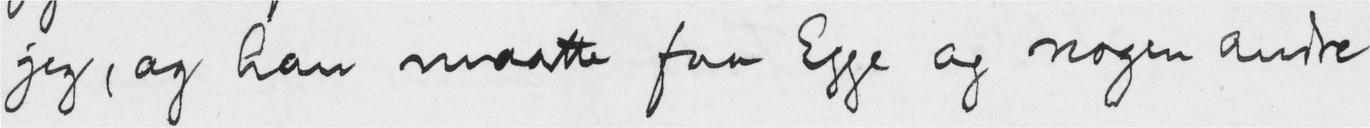jeg, og han maatte faa Egge og nogen andre
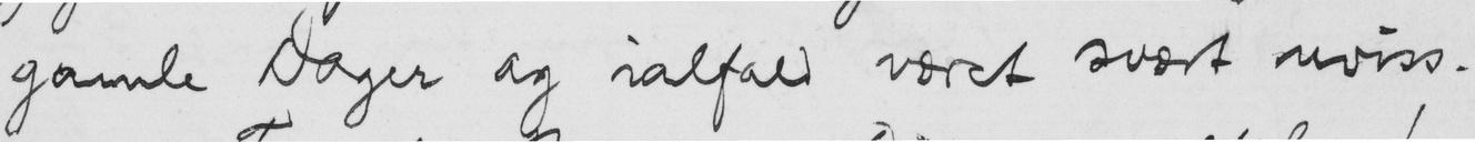gamle Dager og ialfald været svært uviss.
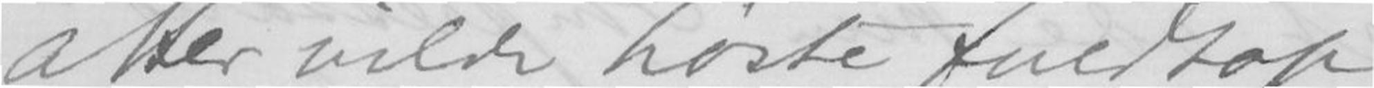atter vilde høste fuldtop
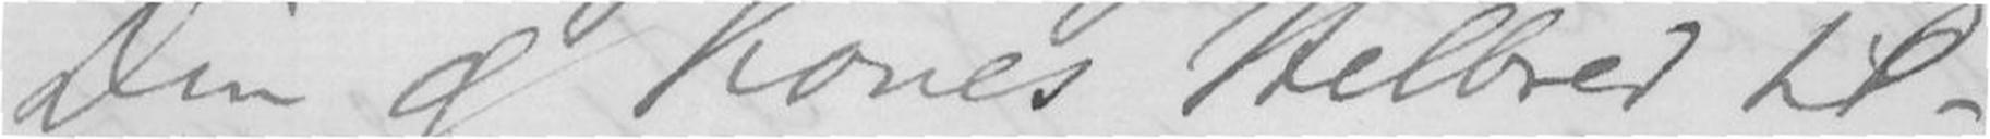Din og Kones Helbred til-
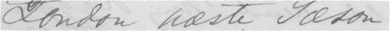London næste Sæson -
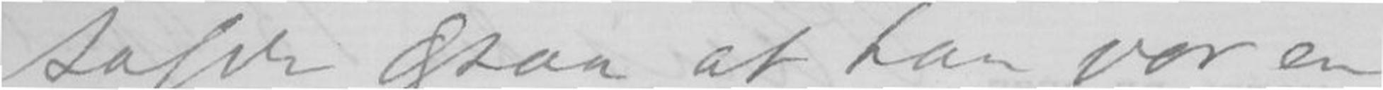sagde ogsaa at han var en
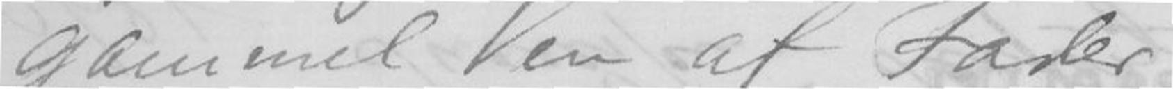gammel Ven af Fader.
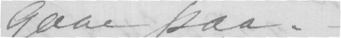gaae paa. -
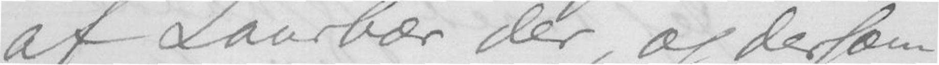af Laurbær der, og dersom
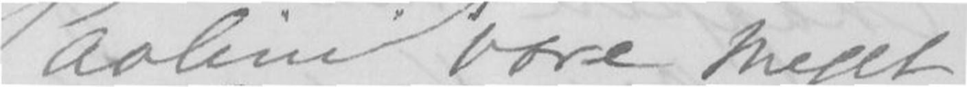Paolinis være meget
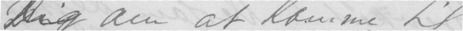Dig om at komme til
